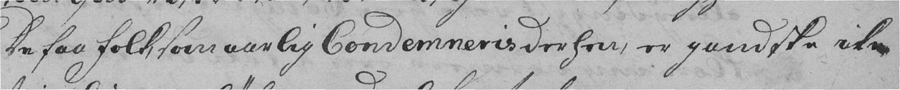De faa folk som aarlig Condemneris derhen, er gandske ikke
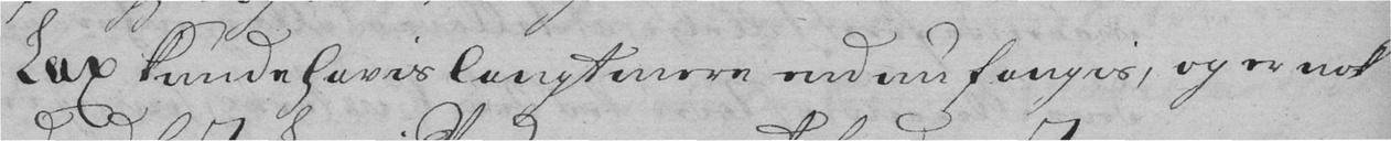Lax kunde havis langt mere end nu fangis, og er nok
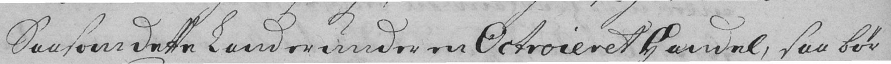Saa som dette Land er under en Octroieret Handel, saa bør
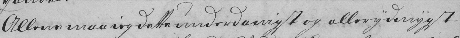Allene maa ieg dette underdanigst og allerydmygst
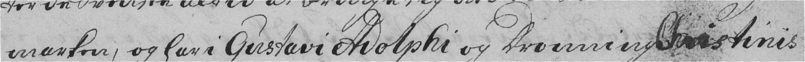marken, og har i Gustavi Adolphi og Dronning Christinis
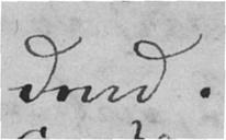dend.
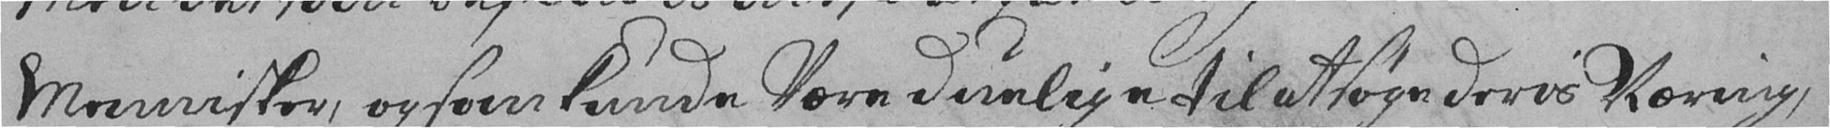Mennisker, og som kunde Være duelige til at søge deres Næring,
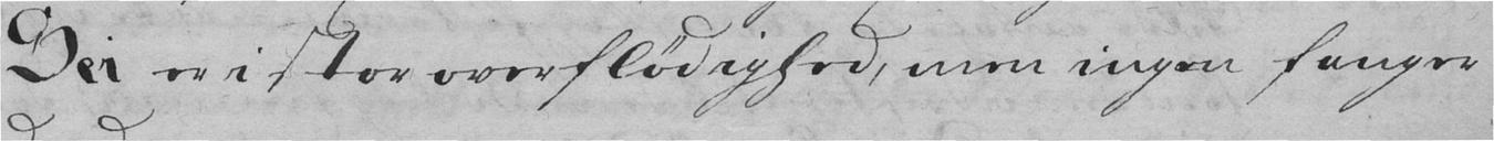Sei er i stor overflødighed, men ingen fanger
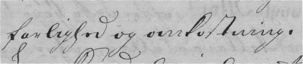farlighed og omkostning.
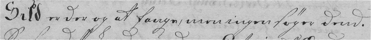Sild er der og at fange, men ingen søger dend.
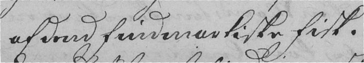af dend Findmarkiske Fisk.
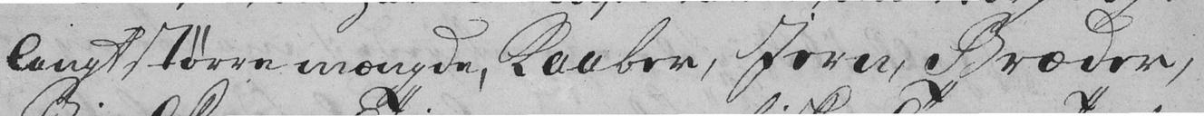langt større mængde, Kaaber, Iern, Bræder,
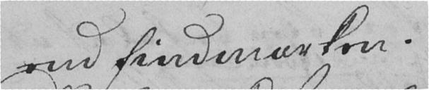end Findmarken.
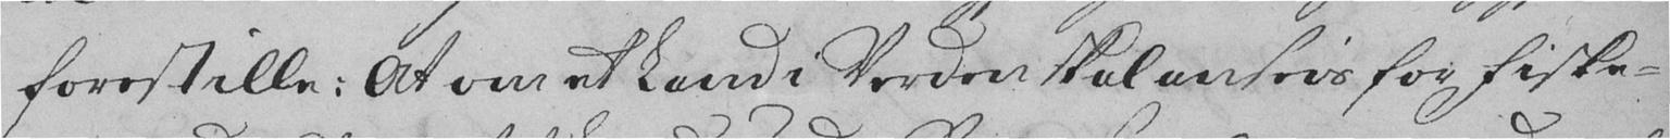forestille: At om et Land i Verden skal anseis for Fiske-
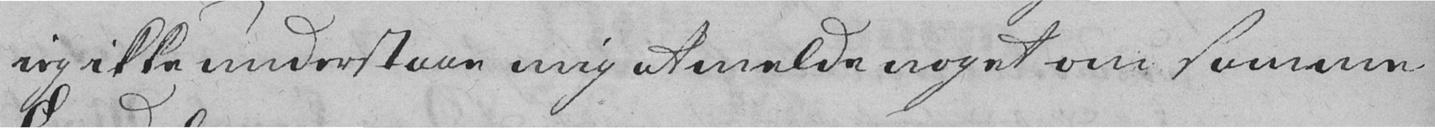ieg ikke understaae mig at melde noget om samme
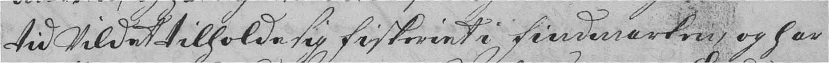tid Vildet tilholde sig Fiskeriet i Findmarken, og har
